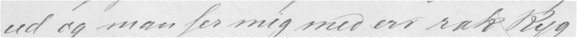ud og man ser mig med en rak Ryg
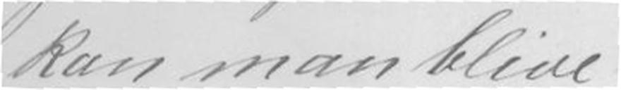kan man blive
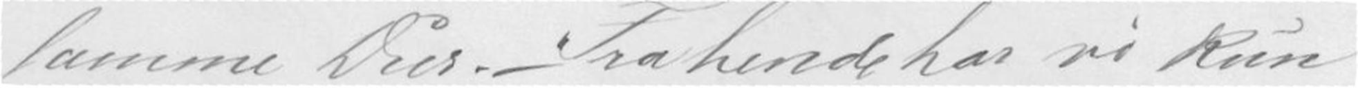samme Dur.- Fra hende har vi kun
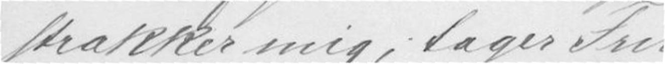strakker mig, tager Fre
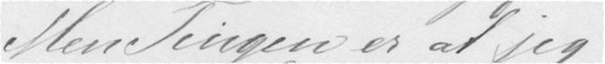Men Tingen er at jeg
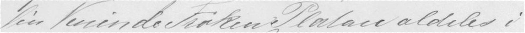sin Veninde Frøken Plalace aldeles i
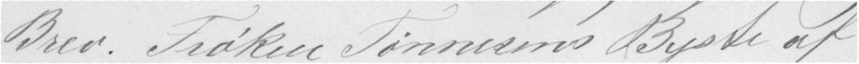Brev. Frøken Sønnermos af
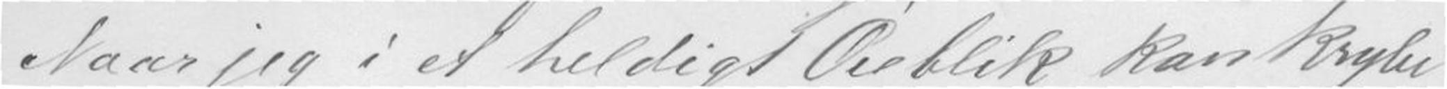Naar jeg i et heldigt Øieblik kan krybe
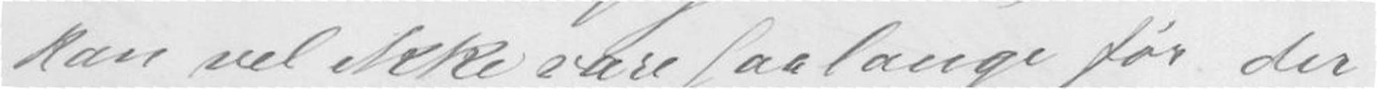kan vel ikke være saalænge før der
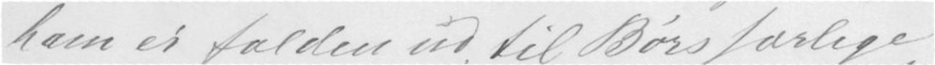havn er falden ud til Børs/ ærlege
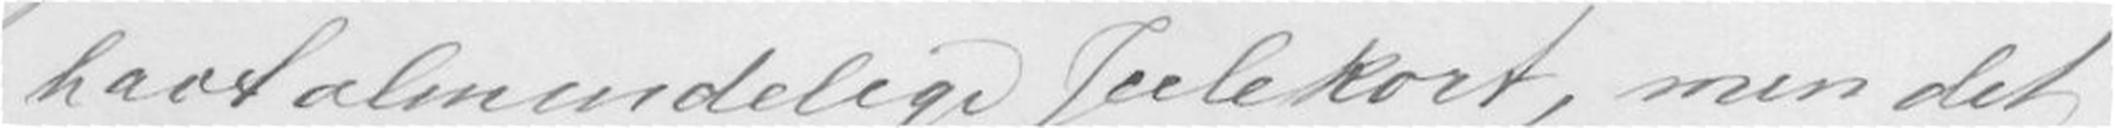havt almindelige Julekort, men det
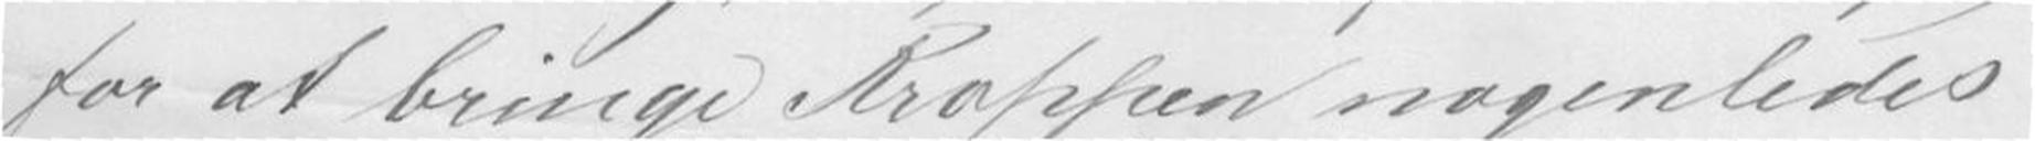for at bringe Kroppen nogenledes
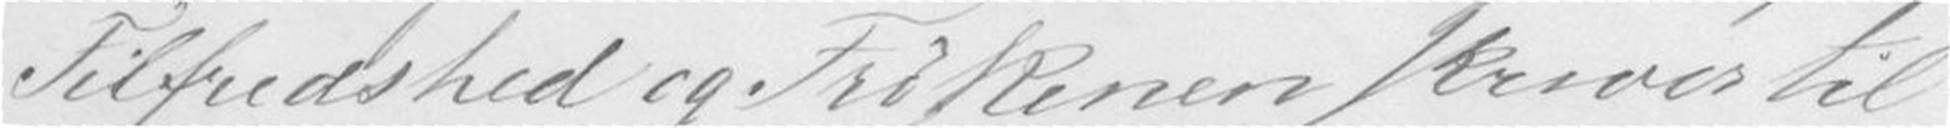Tilfredshed og Frøkenen til
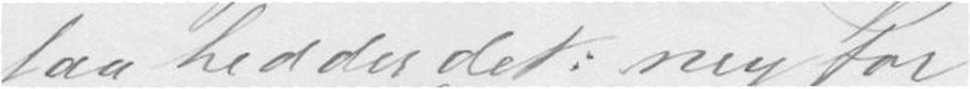faa hedder det my for
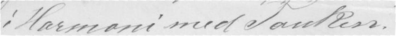i Harmoni med Tanken.
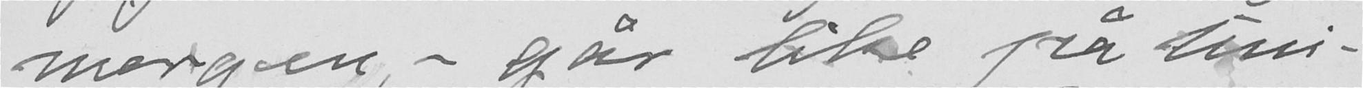morgen - går like på uni-
